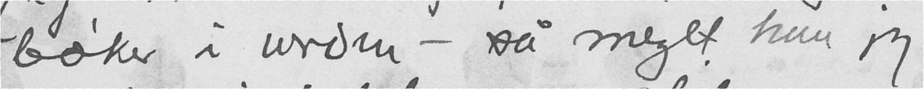bøker i verden - så meget kan jeg
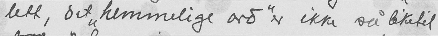lett, det "hemmelige ord" er ikke så liketil
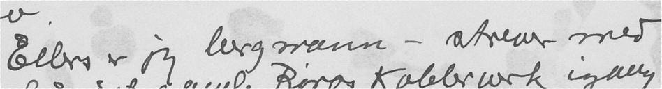Ellers er jeg bergmann - strever med
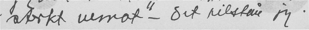strkt besnot - Det tilstår jeg.
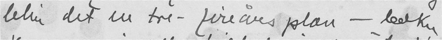blir det en tre - fireårs plan - boken
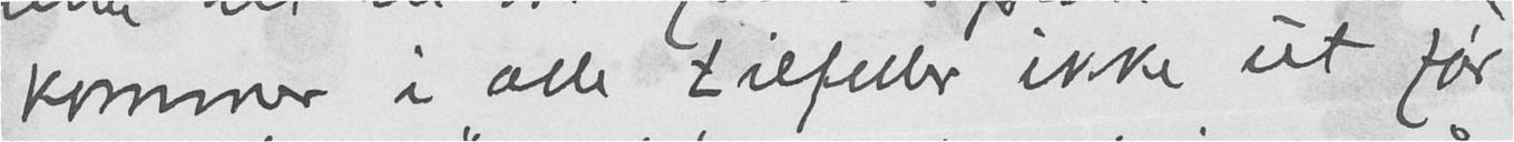kommer i alle tilfeller ikke ut før
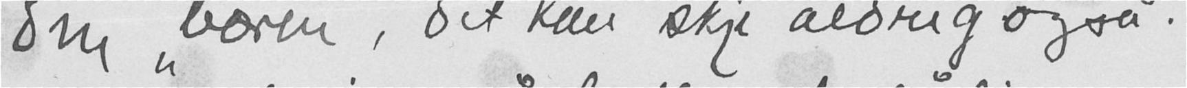om "boren". Det kan skje aldrig også.
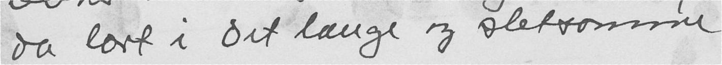da lort i det lange og slitsomme
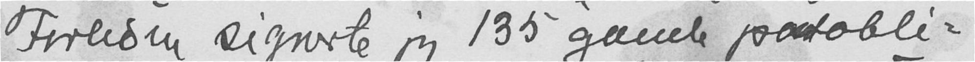Forleden signerte jeg 135 gamle obli-
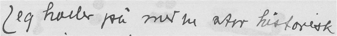Jeg holder på med en stor historisk
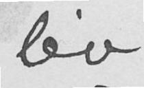liv.
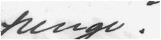penge.
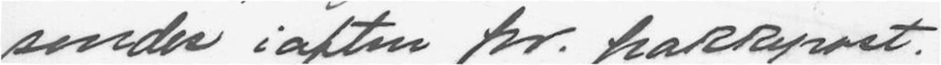sendes iaften pr. pakkepost.
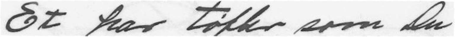Et par tøfler som Du
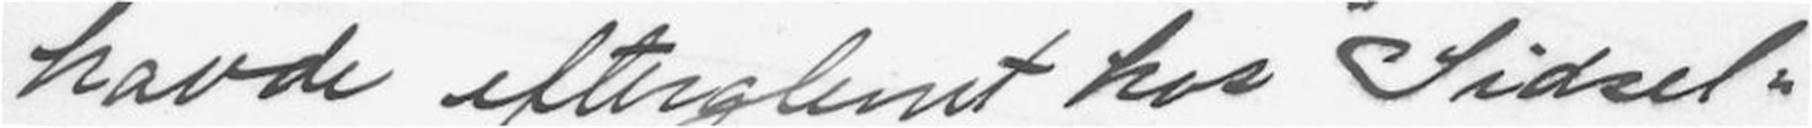havde efterglemt hos "Sidsel"
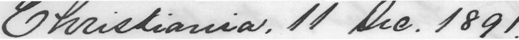Christiania. 11 Dec. 1891.
